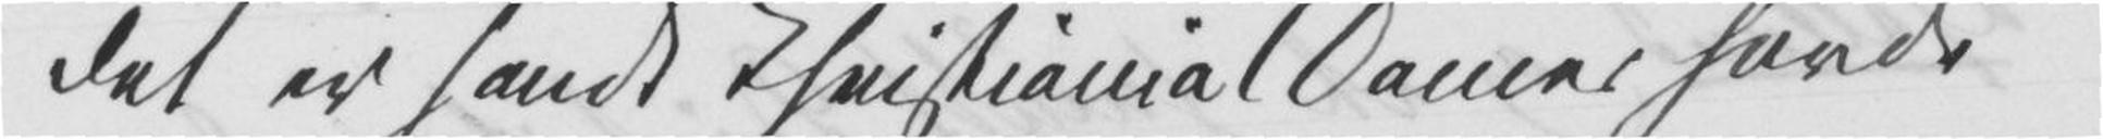det er sandt Christiania Damer havde
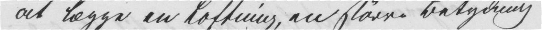at lægge en Løftning, en større Betydning
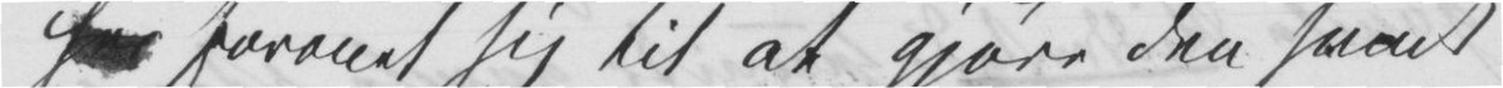her forenet sig til at gjøre den smuk
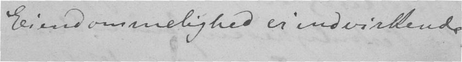Eiendommelighed er indvirkende
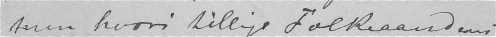ten hvori tillige Folkeaandens
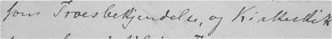som Troesbekjendelse, og Kirkeskik
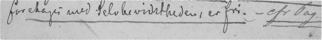foretages med Selsbevidstheden, er fri. – cfr Pag.
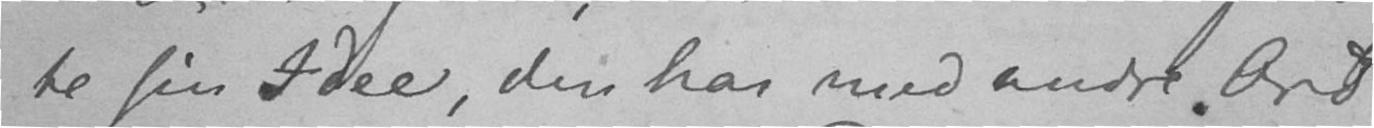te sin Idee, den har med andre Ord
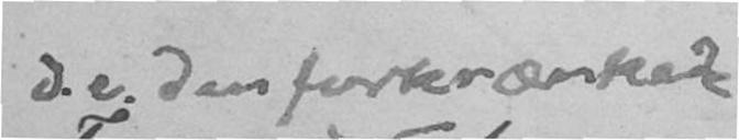d.e. den forkrænkede
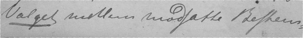Valget mellem modsatte Bestem-
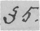§ 5.
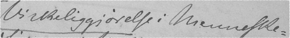Virkeliggjørelse i Menneske-
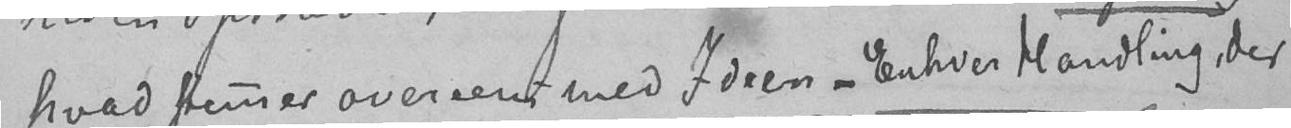hvad stemmer overeens med Ideen – Enhver Handling, der
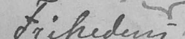Frihedens
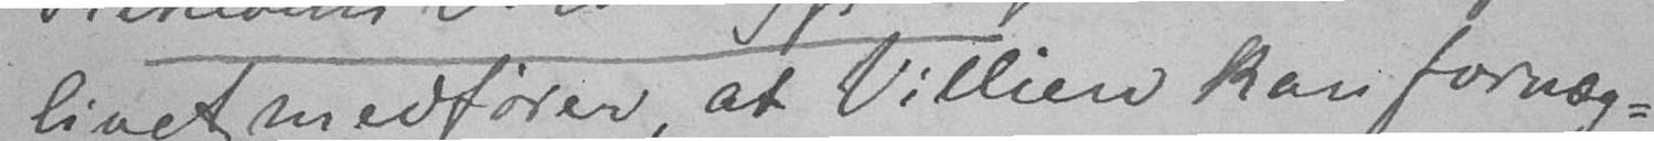livet medfører, at Villien kan fornæg-
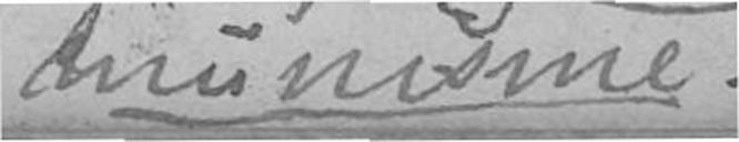munisme.
